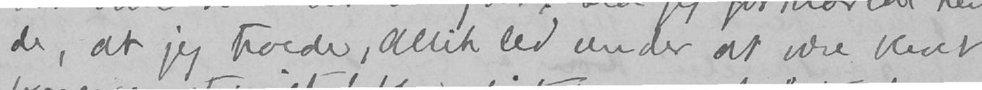de, at jeg troede, Allik led under at være blevet
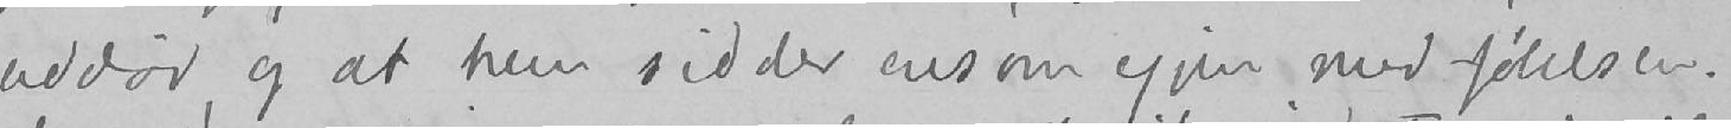uddød, og hun sidder ensom igjen med følelsene.
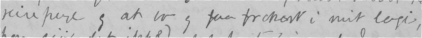reisepenge og at bo og faa frokost i mit logi,
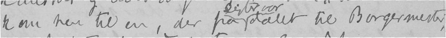kom hen til en, der fra dalet til Borgermester,
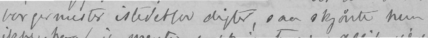borgermester istedetfor digter, saa skjønte hun
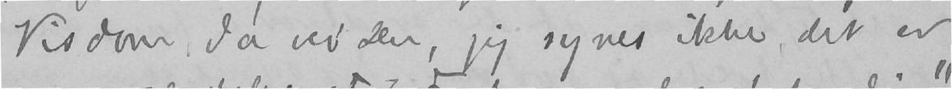Visdom, Ja ved Du, jeg synes ikke det er
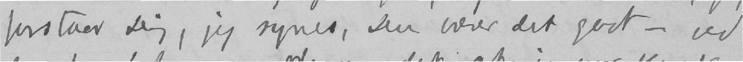forstaar Dig, jeg synes, Du bærer det godt – ved
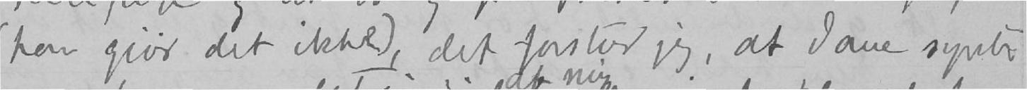(han gjør det ikke), det forstod jeg, at Jane syntes
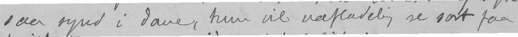saa synd i Jane, hun vil uafladelig se sort paa
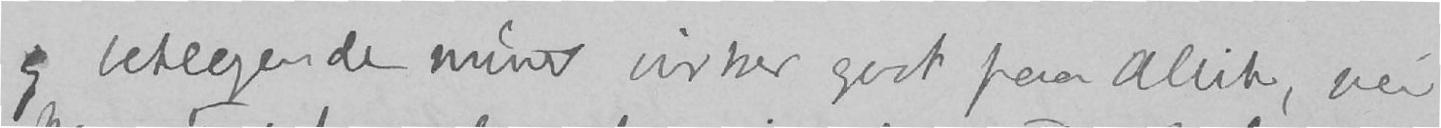og beklagende miner virker godt paa Allik, nei
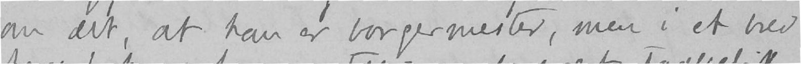om det, at han er borgermester, men i et brev
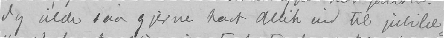Jeg vilde saa gjerne havt Allik ind til jubilæ-
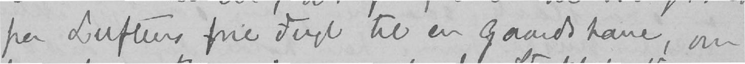fra Luftens frie Fugl til en Gaardshane, om
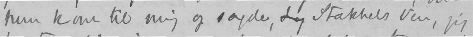hun kom til mig og sagde, jeg Stakkels Ven, jeg
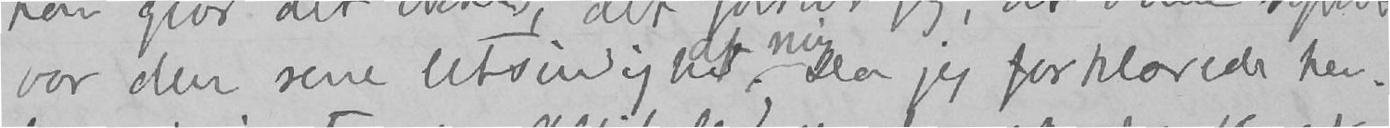var den rene letsindighet af mig. Da jeg forklarede hen-
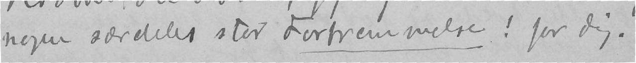nogen særdeles stor Forfremmelse! for dig.»
